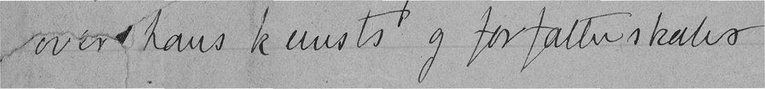over hans kunsts og forfatterskabs
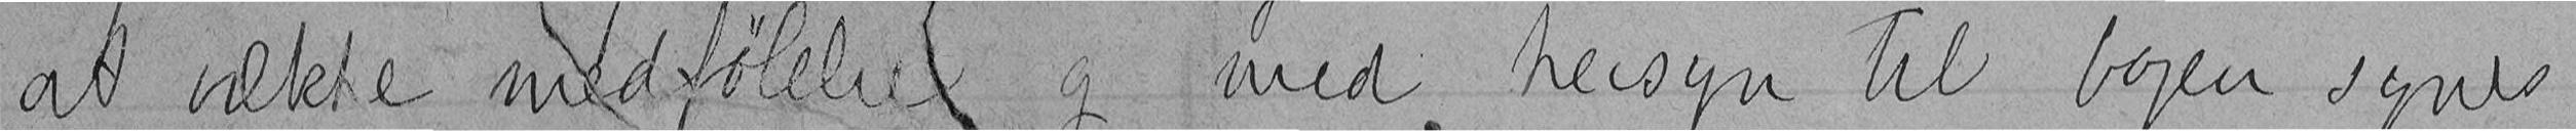at vække medfølelse og med hersyn til bogen synes
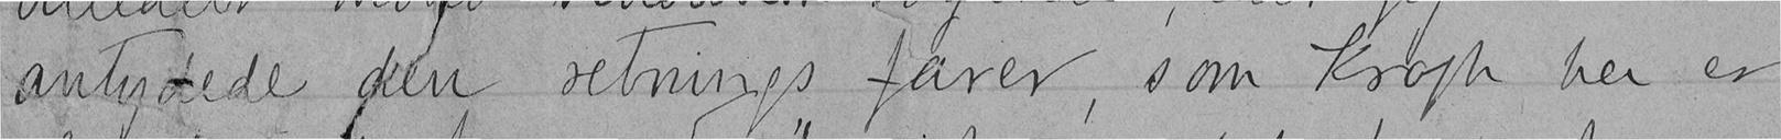antydede den retnings farer, som Krogh her er
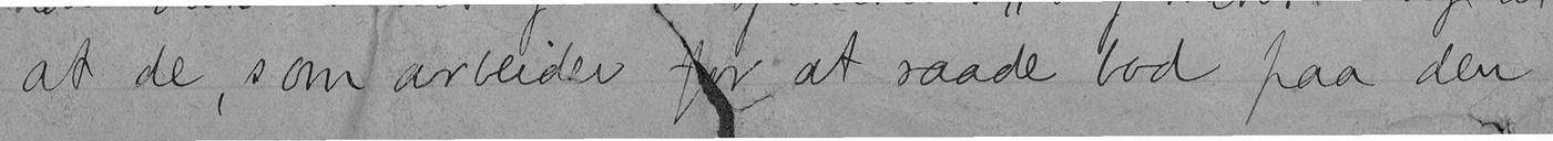at de, som arbeider for at raade bod paa den
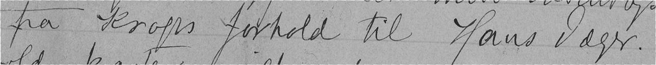fra Kroghs forhold til Hans Jæger.
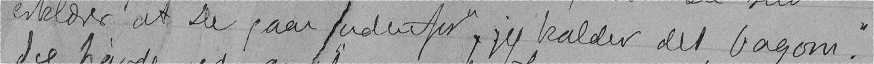erklærer at De gaar udenfor, "jeg kalder det bagom."
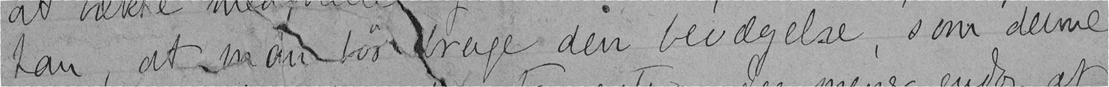han, at man bør drage den bevægelse, som denne
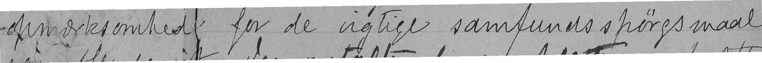opmærksomhed for de vigtige samfundsspørgsmaal
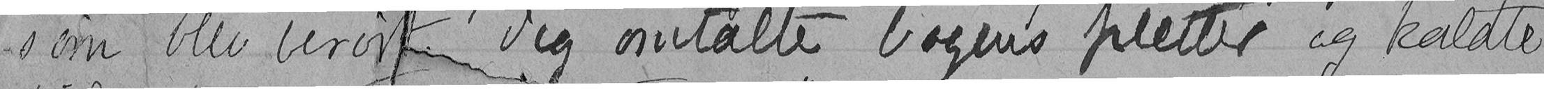som blev berørt. Jeg omtalte bogens pletter og kaldte
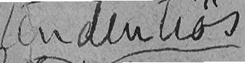tendentiøs
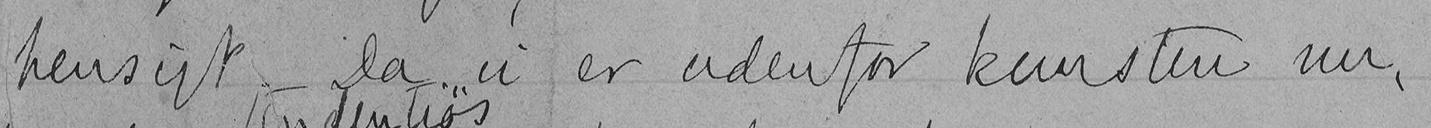hensigt. Da vi er udenfor kunsten nu,
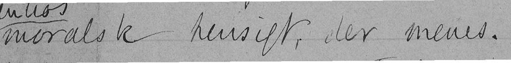moralsk hensigt, der menes.
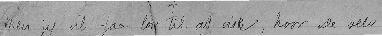Men jeg vil faa log til at vise, hvor De selv
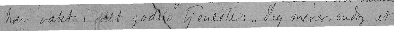har vakt i det godes tjeneste. "Jeg mener endog at
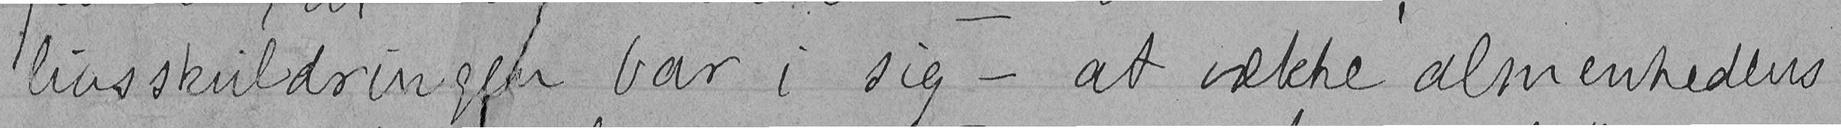livsskildringen bar i sig - at vække almenhedens
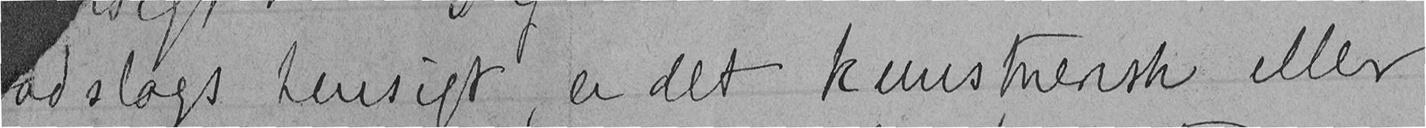adslags hensigt, er det kunstnerisk eller
